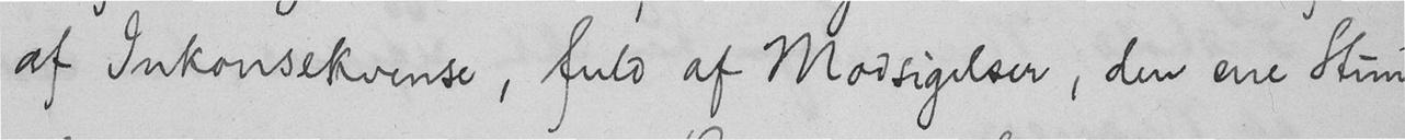af Inkonsekvense, fuld af Modsigelser, den ene Stun
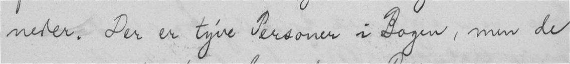neder. Der er tyve Personer i Bogen, men de
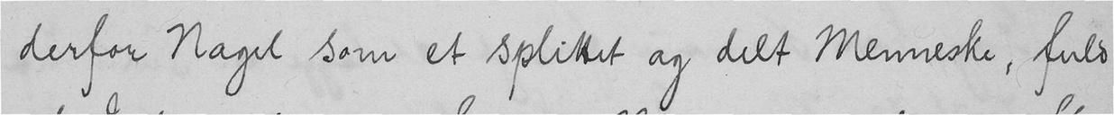derfor Nagel som et splittet og delt Menneske, fuld
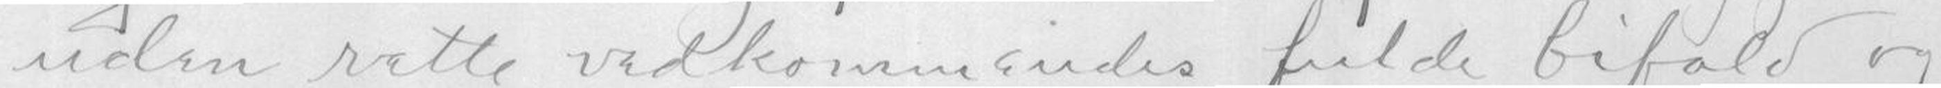uden rette vedkommende fulde bifald og
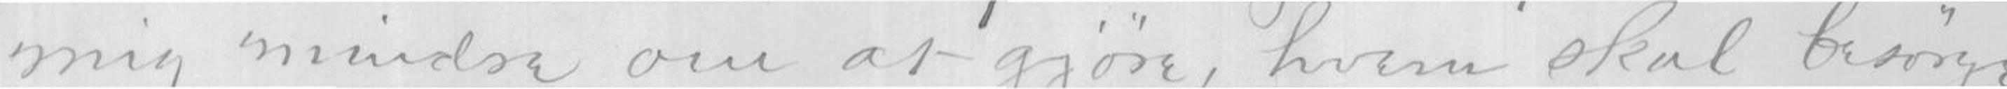mig mindre om at gjøre, hvem skal besørge
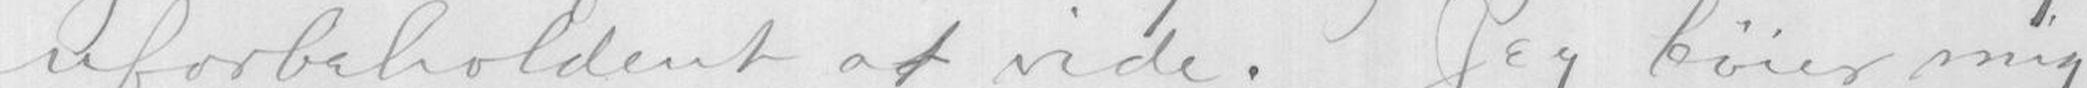uforbeholdent af vide. Jeg bøier mig
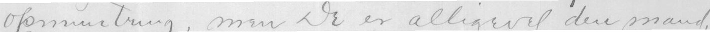opmuntring, men De er alligevel den mand,
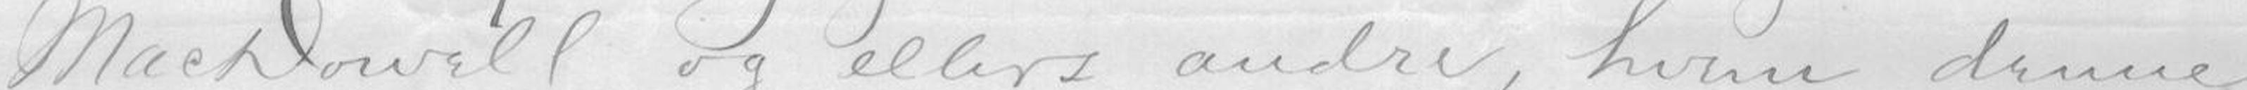MacDowell og ellers andre, hvem denne
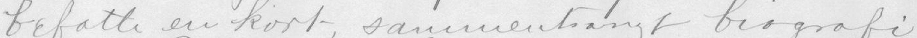befatte en kort, sammentrengt biografi
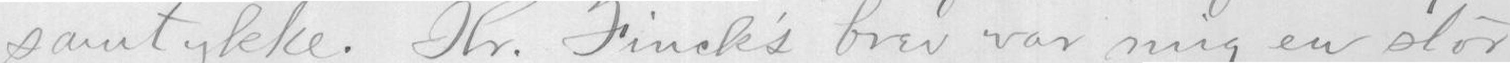samtykke. Hr. Finck's brev var mig en stor
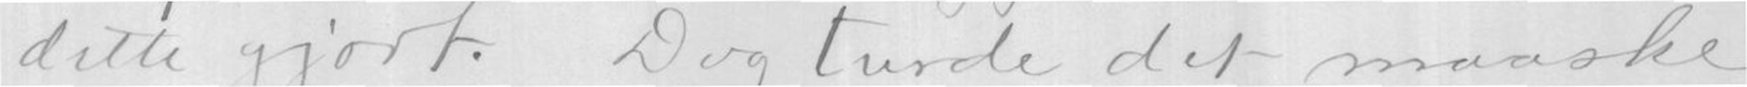dette gjort. Dog burde det maaske
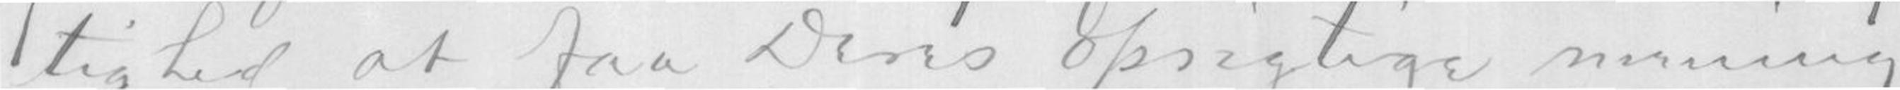tighed at faa Deres oprigtige mening
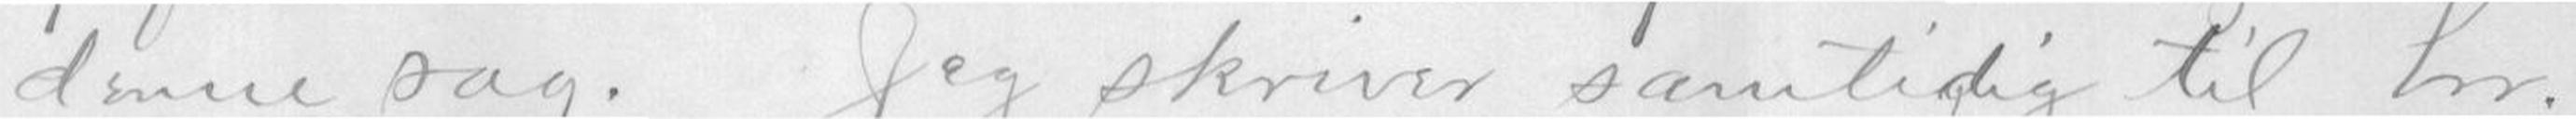denne sag. Jeg skriver samtidig til Hr.
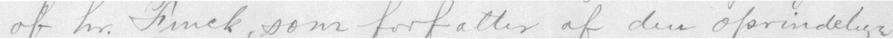af hr.Finck, som forfatter af den oprindelige
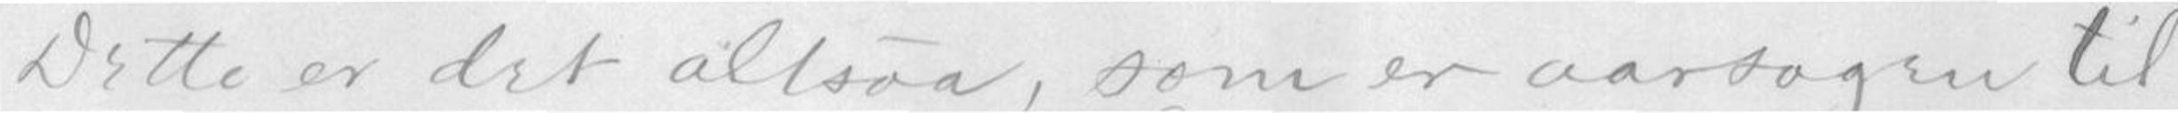Dette er det altsaa, som er aarsagen til
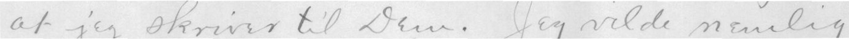at jeg skriver til Dem. Jeg vilde nemlig
